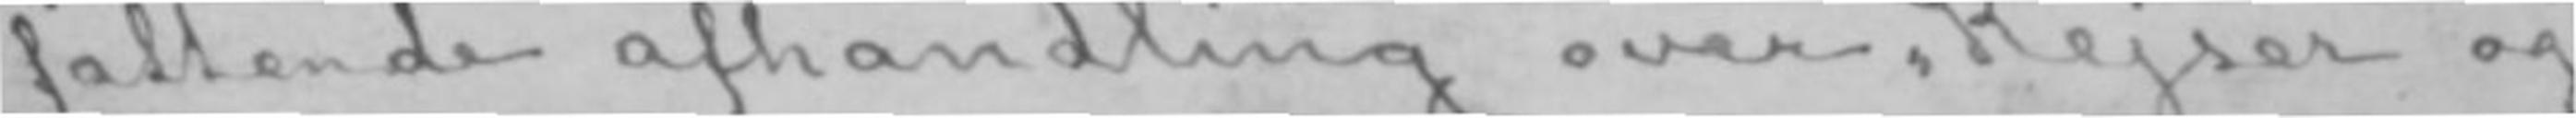fattende afhandling over «Kejser og
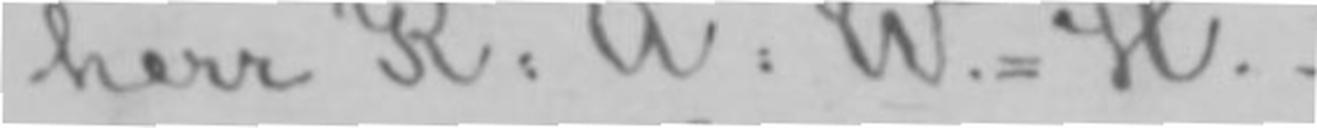herr K: A: W.-H.-
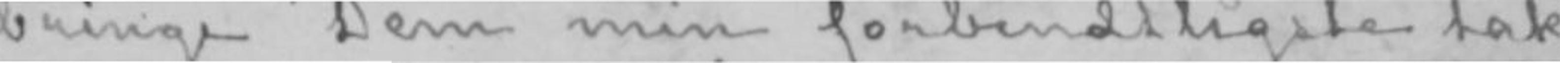at bringe Dem min forbindtligste tak
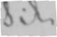Til
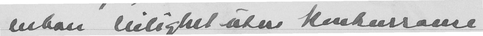enhver leilighet uten konkurranse
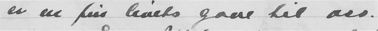er en fin livets gave til oss.
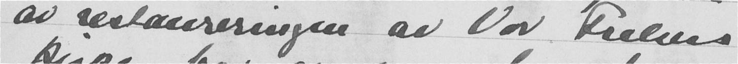av restaureringen av Vor Frelsers
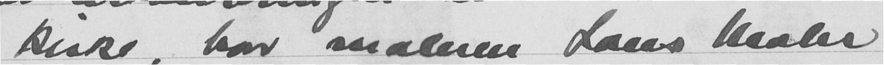kirke, hvor maleren Lars Maler
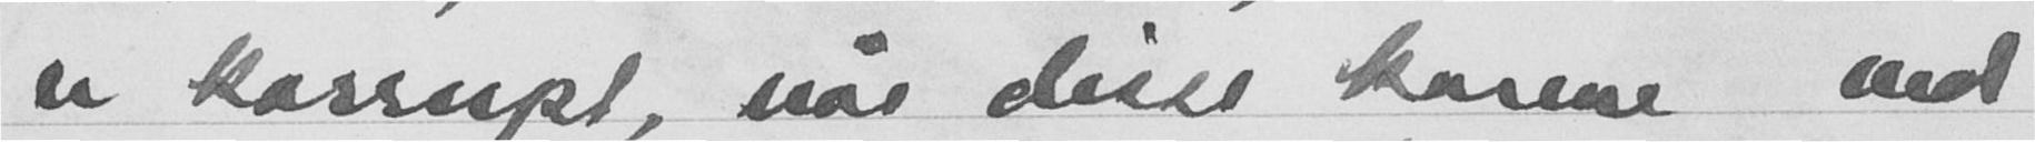er korrupt, nar disse karene ved
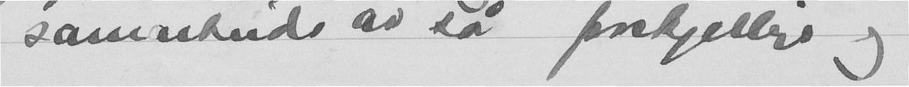samarbeide er så forskjellig og
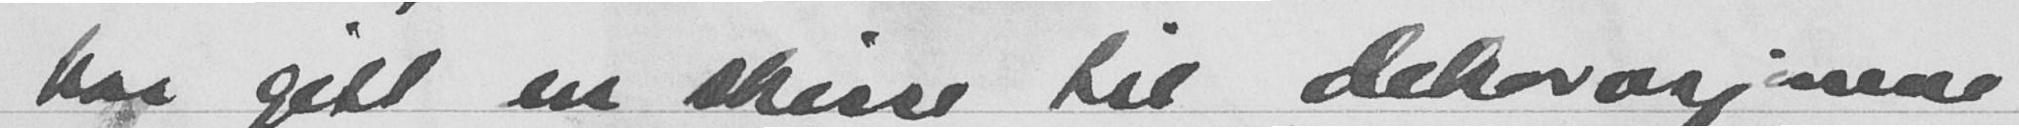har gitt en skisse til dekorasjonene
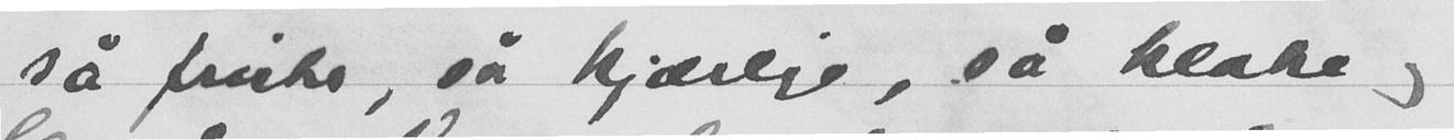så friske, sa kjærlige, så, kloke og
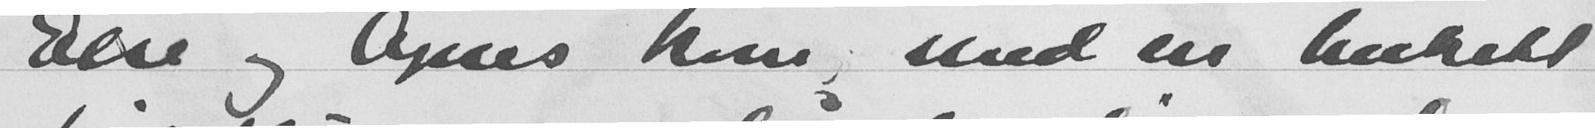Else og Agnes kom med en bukett
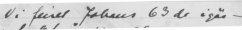Vi feiret Johans 63 de igår -
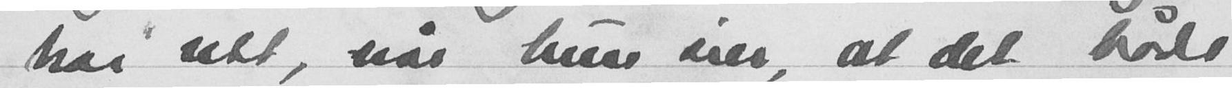har vekt, når hun sier, at det både
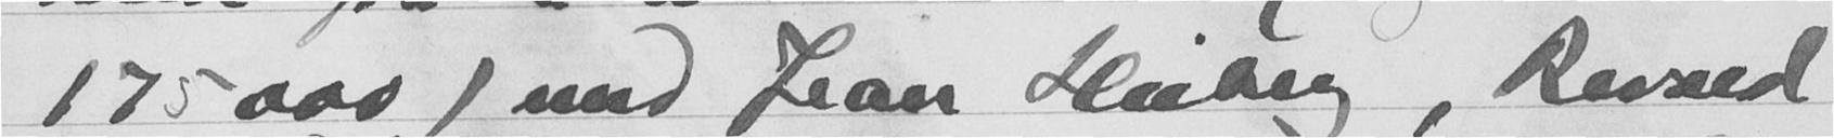175000) med Jean Heiberg, Revold
